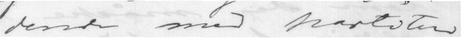dende med partitur,
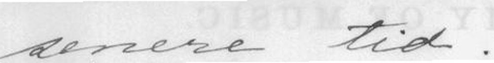senere tid.
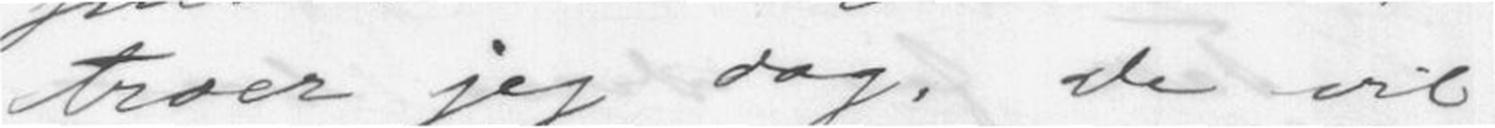troer jeg dog, De vil
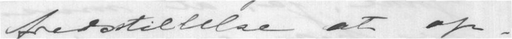fredstillelse at op-
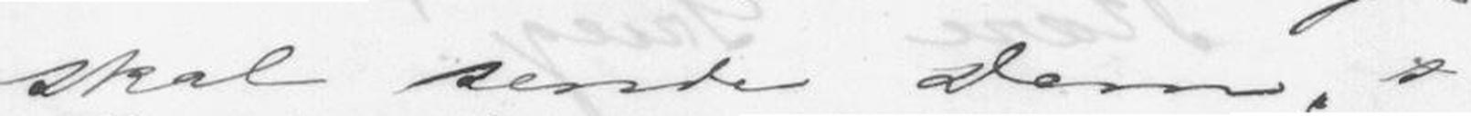skal sende Dem. s
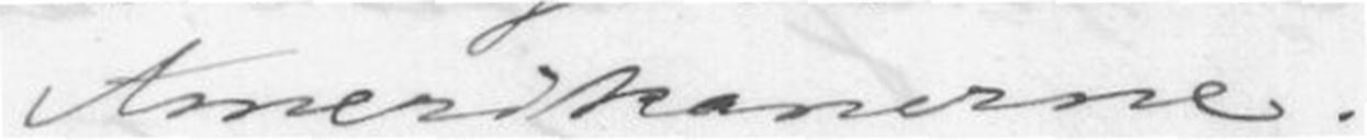Amerikanerne.
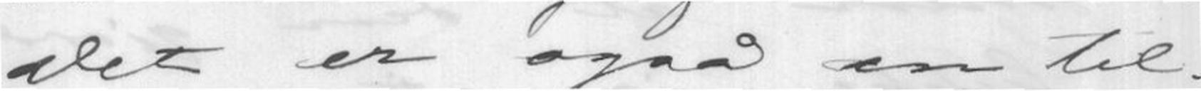Det er også en til-
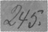245.
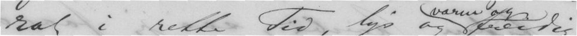rat i rette Tid, lys og freidig
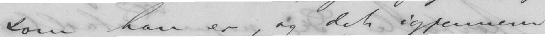som han er, og det igjennem
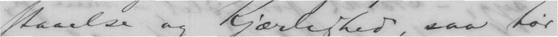staaelse og Kjærlighed, saa tør
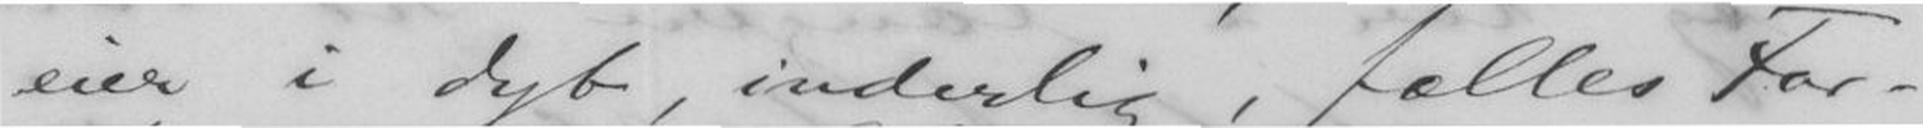eier i dyb, inderlig, fælles For-
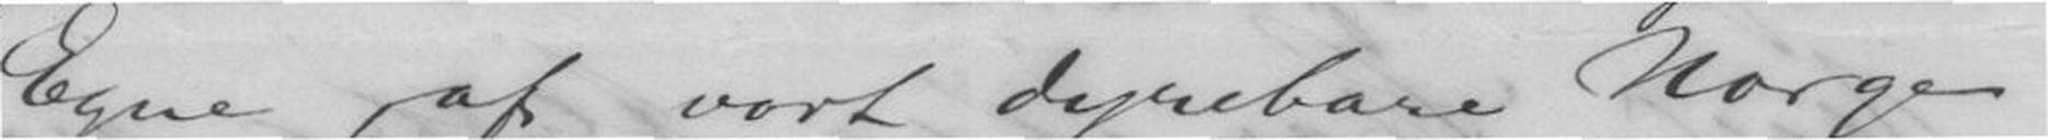Egne af vort dyrebare Norge,
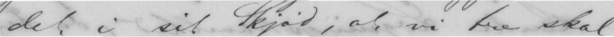det i sit Skjød, at vi tre skal
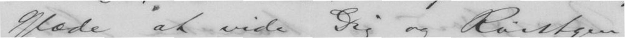Glæde at vide Dig og Röntgen
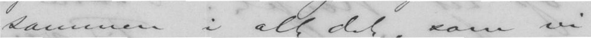sammen i alt det, som vi
